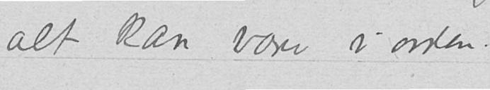alt kan være i orden.
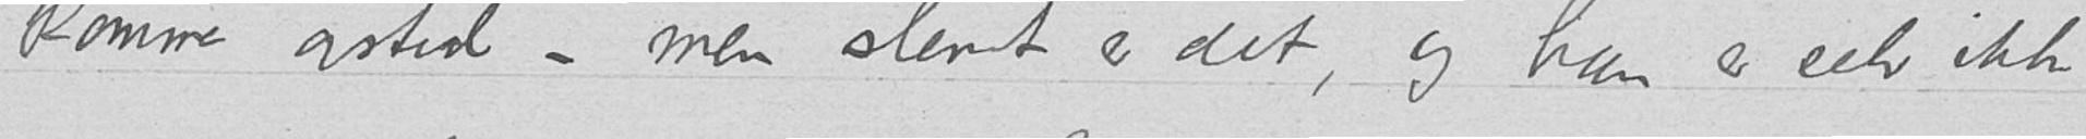komme avsted – men slemt er det, og han er selv ikke
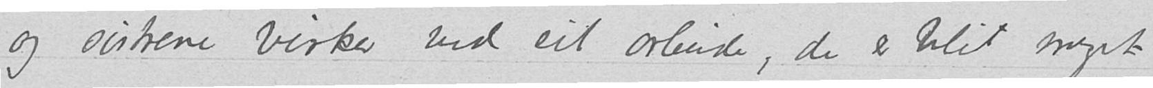og søstrene virker ved sit arbeide, de er blit meget
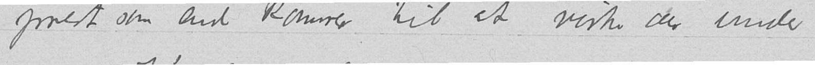prest som end kommer til at virke der under
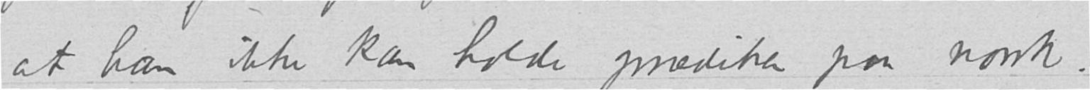at han ikke kan holde prædiken paa norsk.
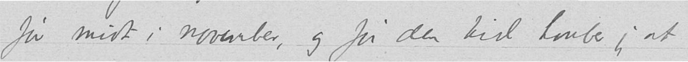før midt i november, og før den tid haaber jeg at
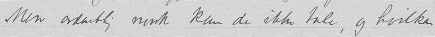Men ordentlig norsk kan de ikke tale, og hvilken
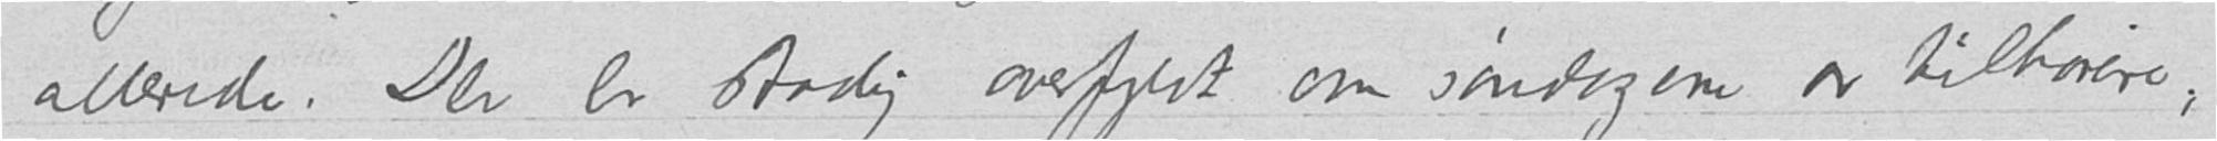allerede. Der er stadig overfyldt om søndagen av tilhørere,
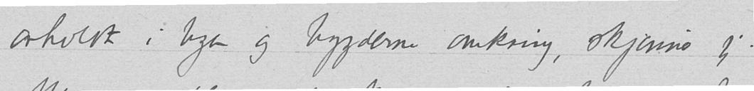avholdt i byen og bygderne omkring, skjønner jeg.
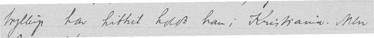bryllup har hittil holdt ham i Kristiania. Men
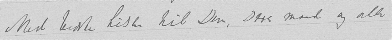Med bedste hilsen til Dem, Deres mand og alle
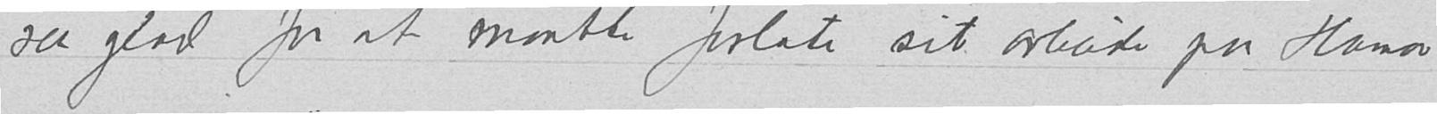saa glad for at maatte forlate sit arbeide paa Hamar
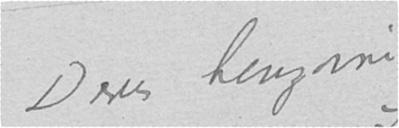Deres hengivne
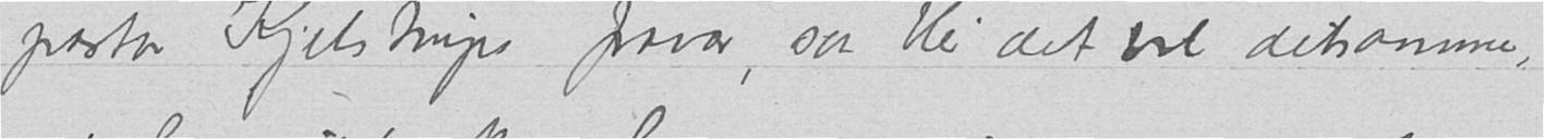pastor Kjelstrups fravær, saa blir det vel detsamme,
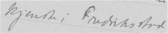kjendte i Fredriksstad
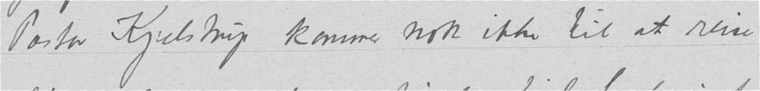Pastor Kjelstrup kommer nok ikke til at reise
